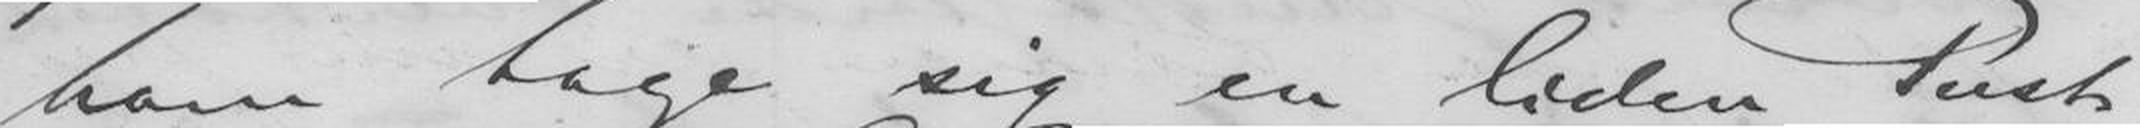ham tage sig en liden Pust
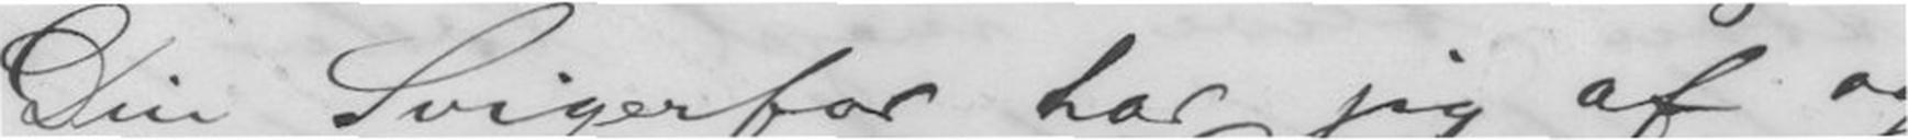Din Svigerfar har jeg af og
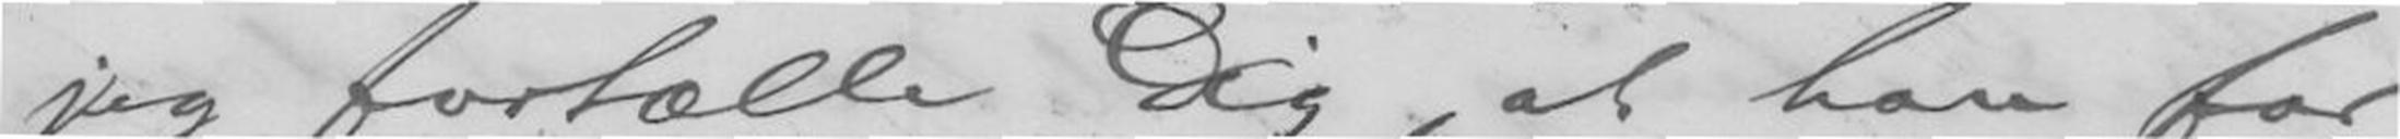jeg fortælle Dig, at han for
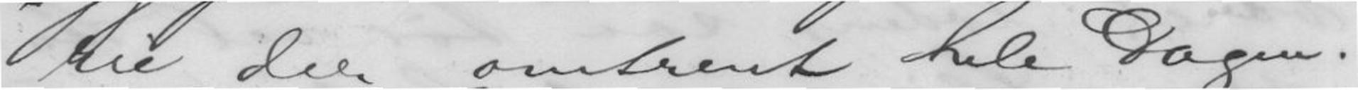rie der omtrent hele Dagen.
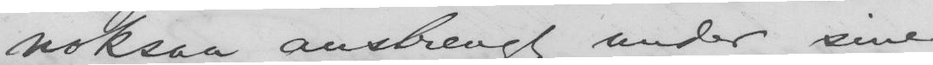noksaa anstrengt under sine
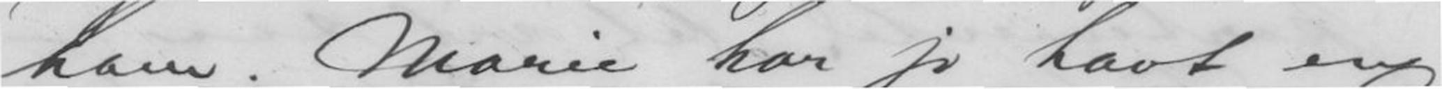ham. Marie har jo havt en
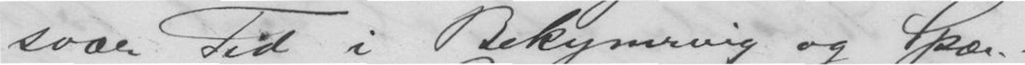vær Tid i Bekymring og Spæn-
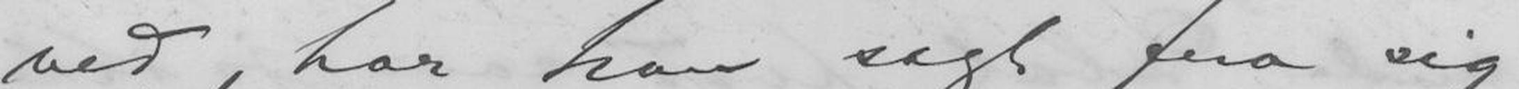ved, har han sagt fra sig
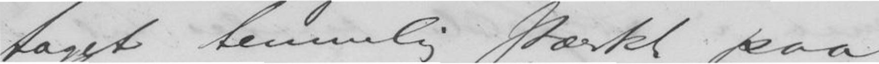taget temmelig stærkt paa
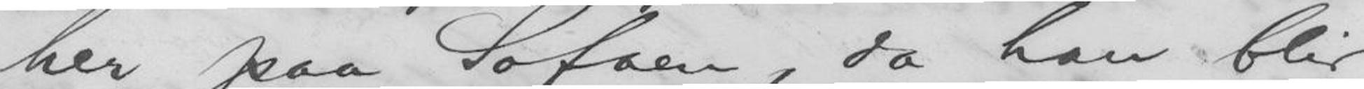her paa Sofaen, da han blir
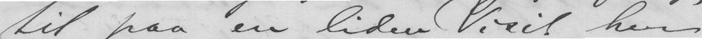til paa en liden Visit her
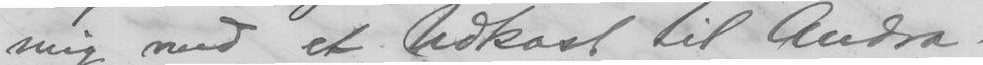mig med et Udkast til Andra-
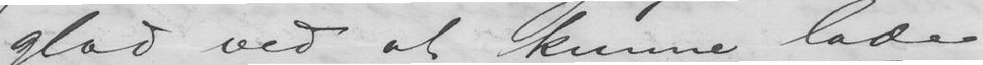glad ved at kunne lade
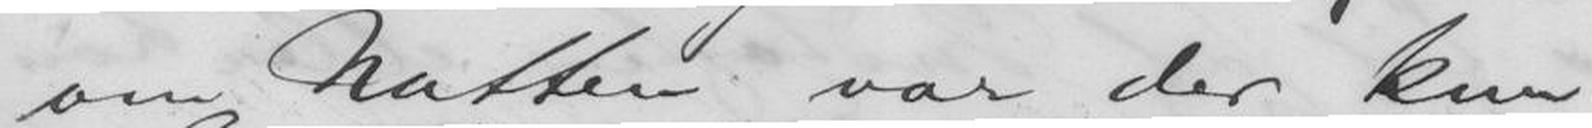om Natten var der kun
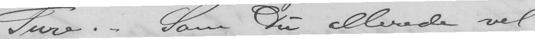Ture. - Som Du allerede vel
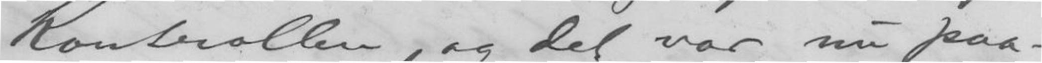Kontrollen, og det var nu paa-
